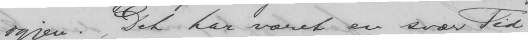igjen. Det har været en svær Tid
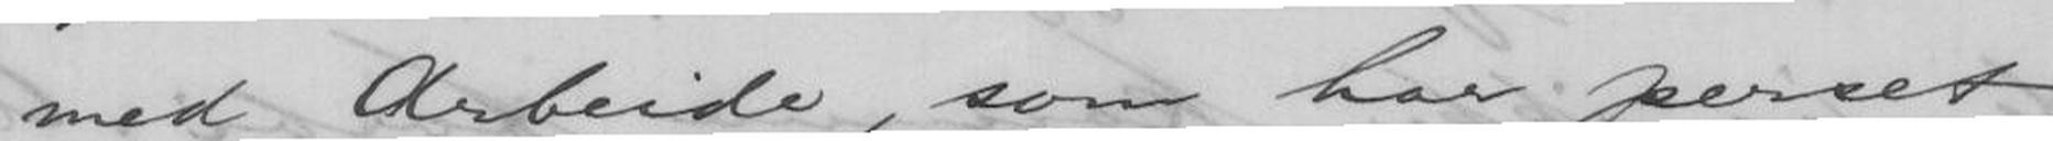med Arbeide, som har perset
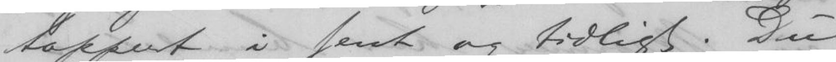tappert i sent og tidligt. Du
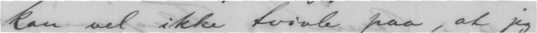kan vel ikke tvivle paa, at jeg
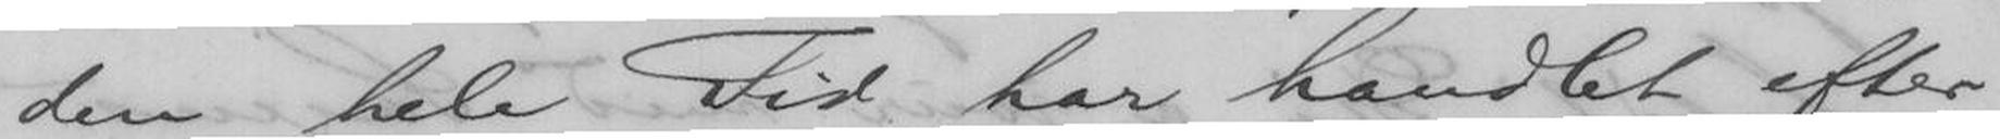den hele Tid har handlet efter
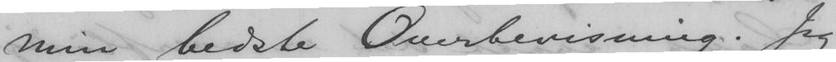min bedste Overbevisning. Jeg
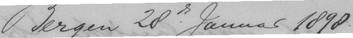Bergen 28de Januar 1898
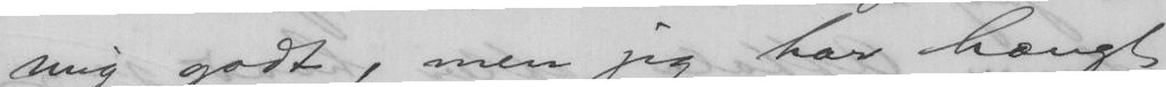mig godt, men jeg har hængt
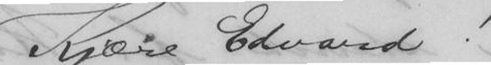Kjære Edvard!
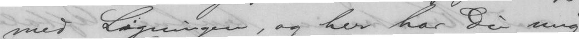med Ligningen, og her har Du mig
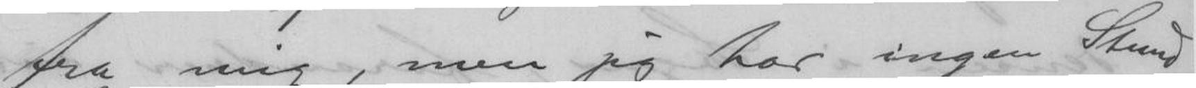fra mig, men jeg har ingen Stund
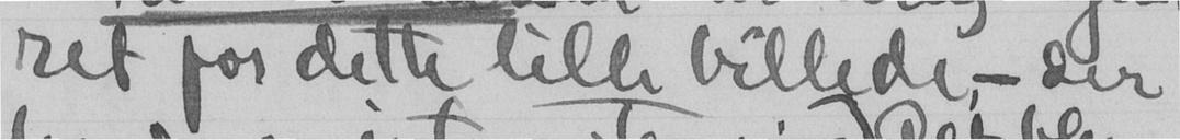ret for dette lille billede, - der
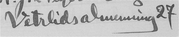Vetrlidsalmenning 27
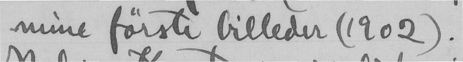mine første billeder (1902).
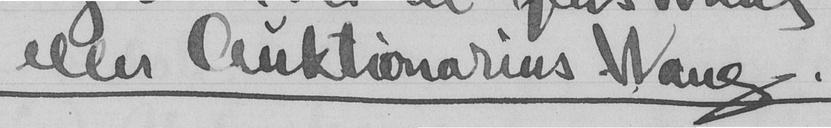eller Auktionarius Wang.
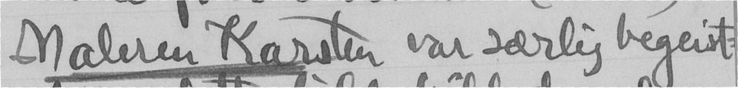Maleren Karsten var særlig begeist-
